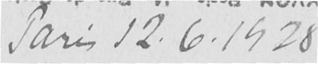Paris 12.6.1928
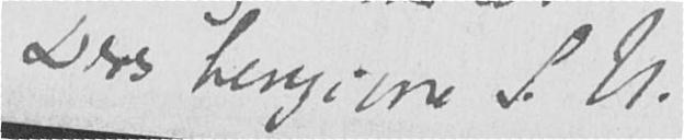Deres hengivne S.U.
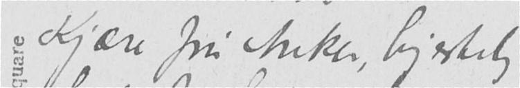Kjære fru Anker, hjertelig
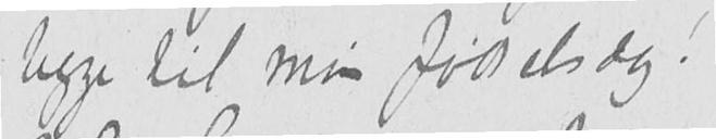begge til min fødselsdag!
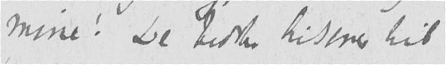mine! De bedste hilsener til
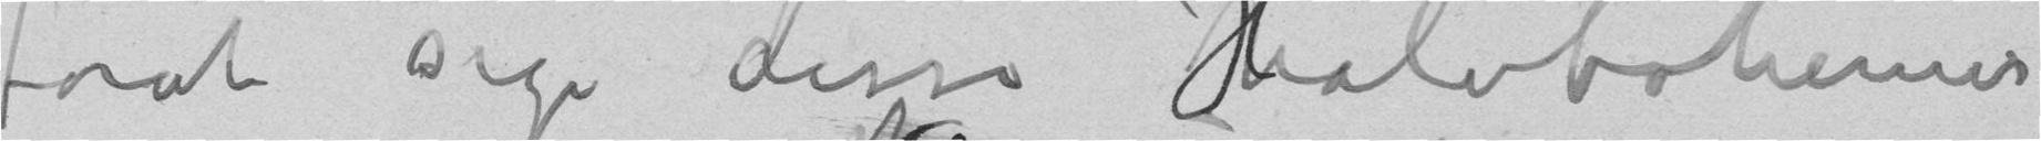forat sige disse Halvbohemer
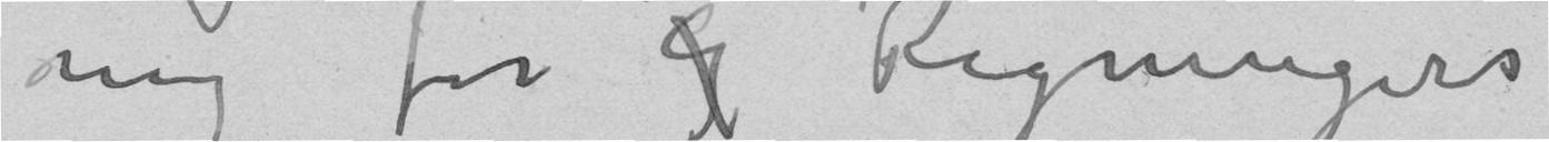mig for g Regnigers
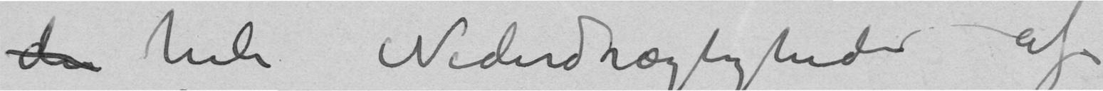den hele Nederdrægtigheder af
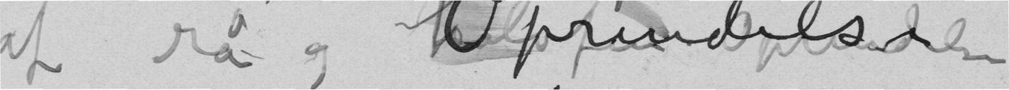af rå og Oprindelse
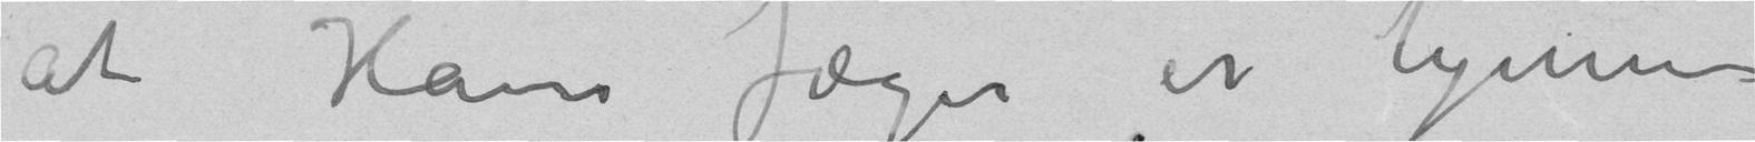at Hans Jæger er hjemme
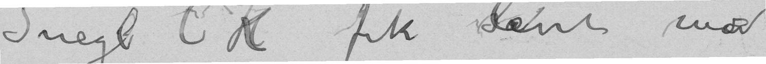Snegl C K fik leve med
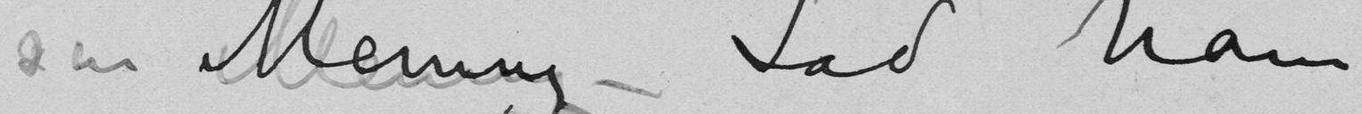sin Mening – Lad ham
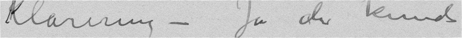Klarering – Ja de kunde
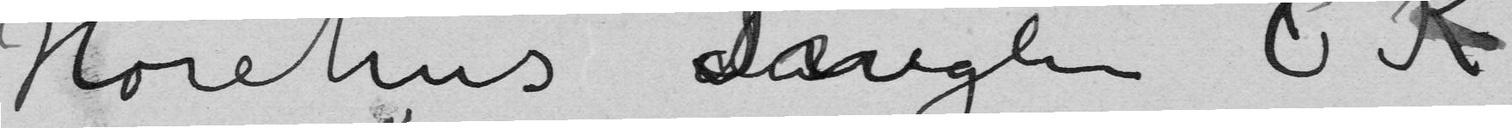Horehus Sneglen C K
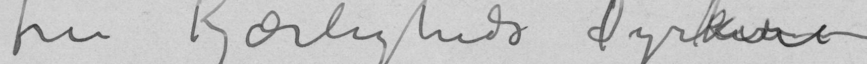fru Kjærligheds Dyrkere
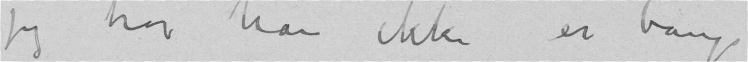jeg tror han ikke er bange
